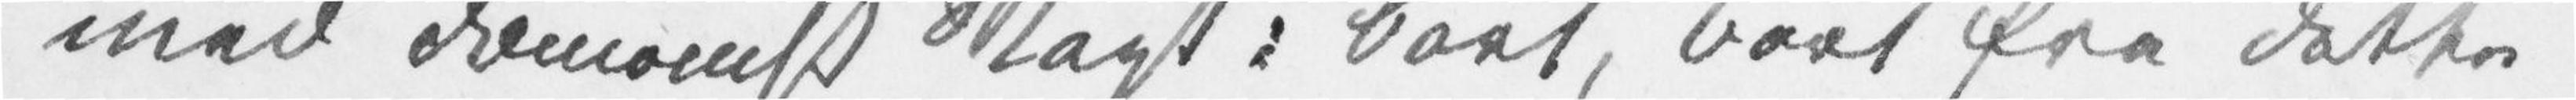med dæmonisk Magt: bort, bort fra dette
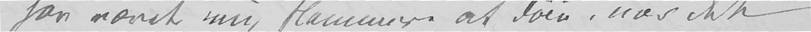har været mig slemmere at døie, når det
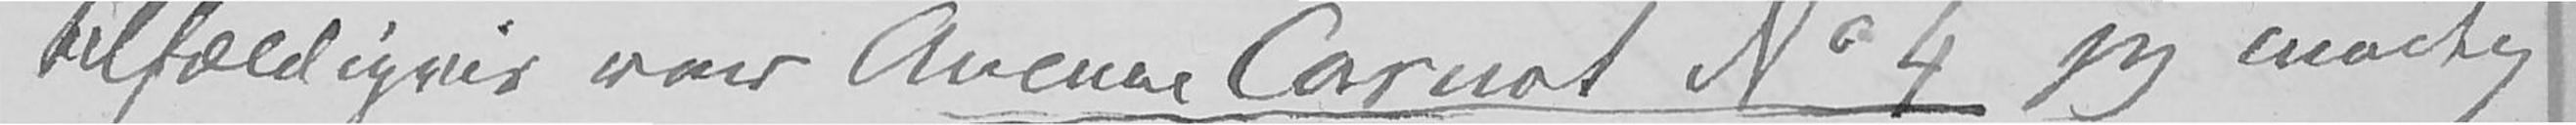tilfældigvis var Avenue Carnot No 4 jeg modtog
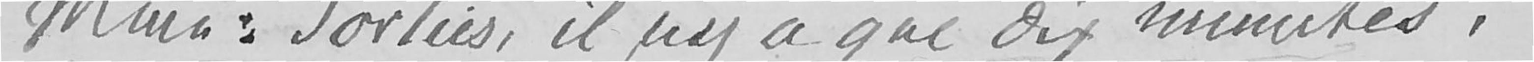Mine: [«]Sorties, il n'y a que dix minutes»,
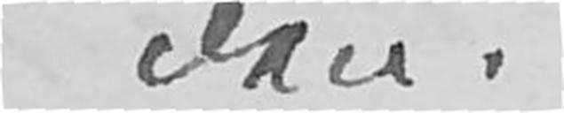den.
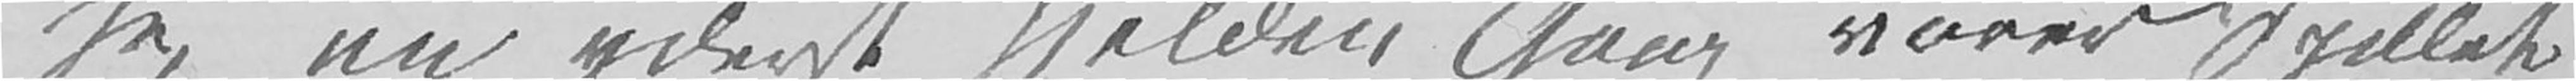jeg en yderst sjelden Gang vover Spillet
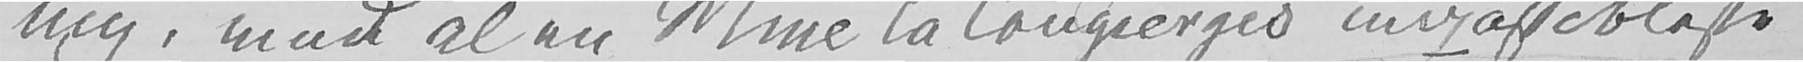mig, med al en Mme la Congiérges impassibleste
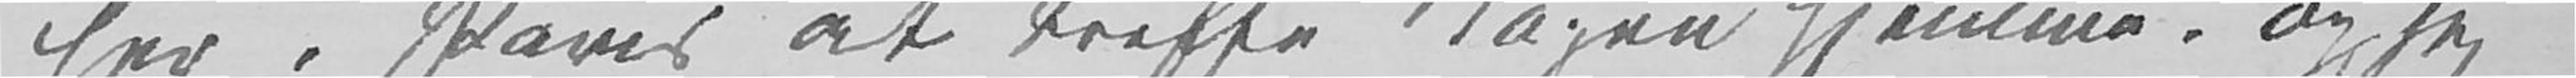her i Paris at treffe Nogen hjemme, og jeg
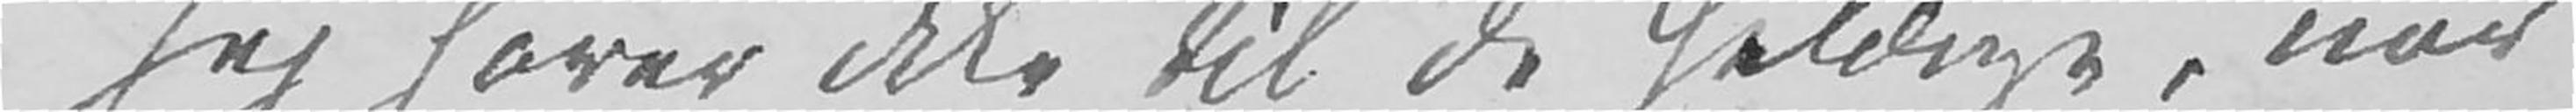Jeg hører ikke til de heldige, når
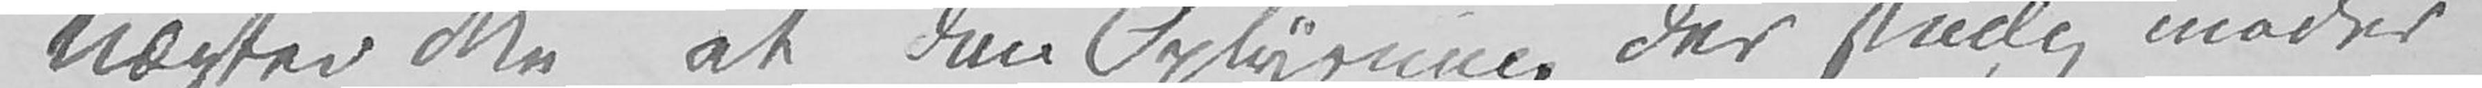nægter ikke, at den Oplysning der stadig møder
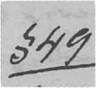§ 49
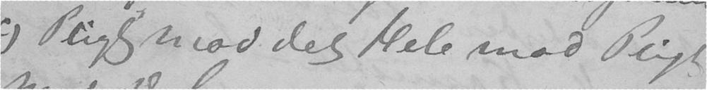c) Pligt mod det Hele mod Pligt
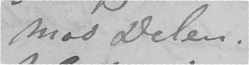mod Delen.
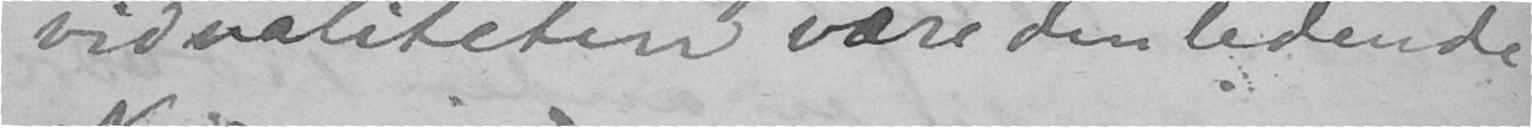vidualiteten være den ledende
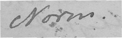Norm.
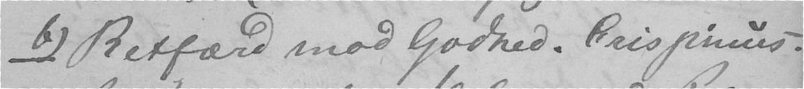b) Retfærd mod Godhed. Prispines.
